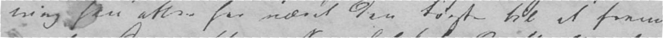ning han atter har været den første til at frem-
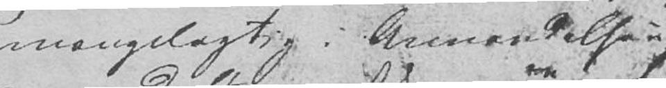mangelagtig i Anvendelsen
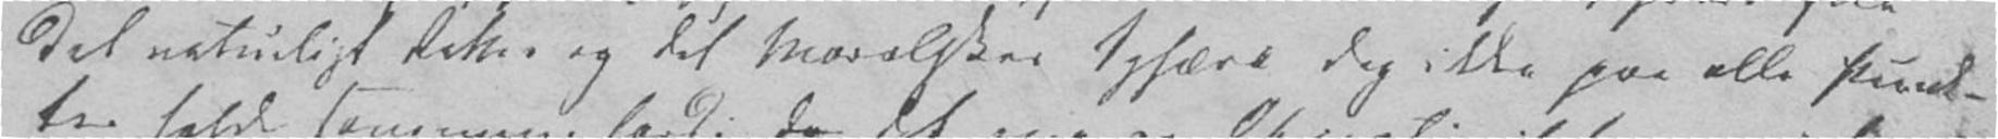det naturligt Rette og det Moralskes Sphære dog ikke paa alle Punk-
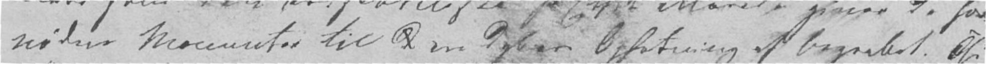nødne Momenter til d en dybere Opfatning af Begrebet. Thi
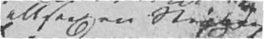altsaa en X
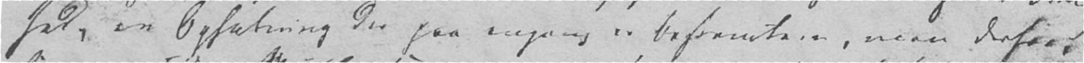hed, en Opfatning der paa engang er bestemtere, men derfor,
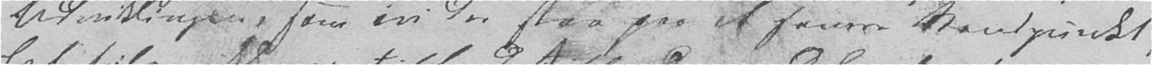Udviklingen, som en der staa paa et senere Standpunkt,
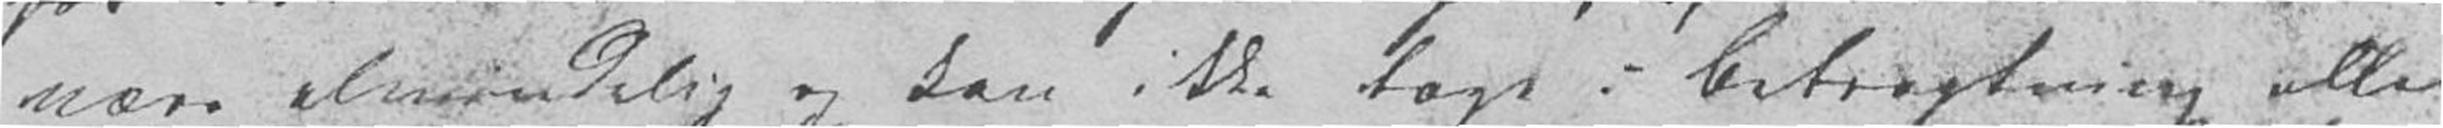være almindelig og kan ikke tage i Betragtning alle
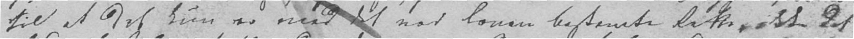til at det kun er med det ved Loven bestemte Rette, ikke det
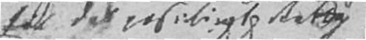X det positivt Rette
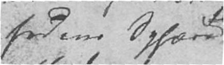hedens Sphære,
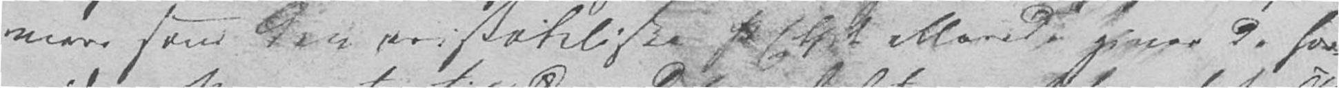mere som den aristoteliske Methode allerede giver de for-
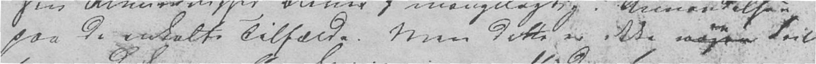paa de enkelte Tilfælde. Men dette er ikke nogen Feil
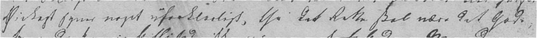Øiekast synes noget uforklarligt, thi det Rette skal være det Gode;
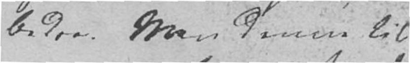bedre. Men denne til-
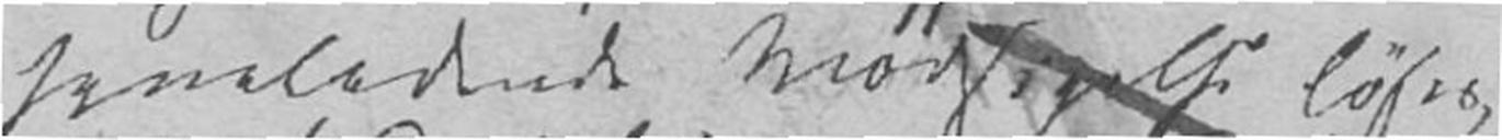syneladende Modsigelse løses,
